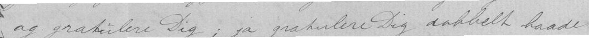og gratulere Dig; ja gratulere Dig dobbelt baade
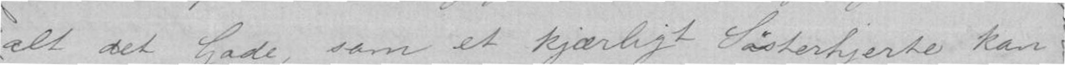alt det Gode, som et kjærligt Søsterhjerte kan
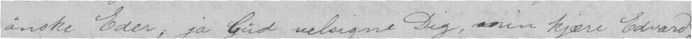ønske Eder; ja Gud velsigne Dig, min kjære Edvard,
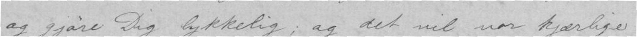og gjør Dig lykkelig; og det vil vor kjærlige
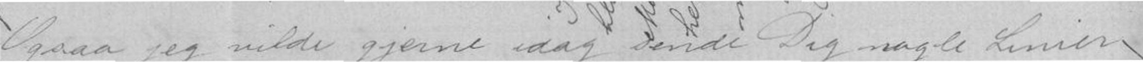Ogsaa jeg vilde gjerne idag sende Dig nogle Linier
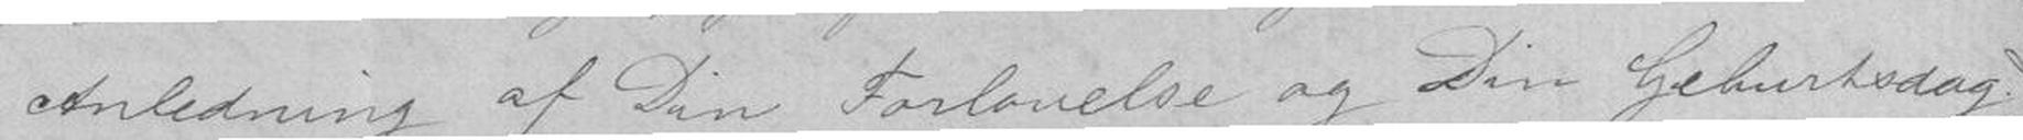i Anledning af Din Forlovelse og Din Gebursdag.
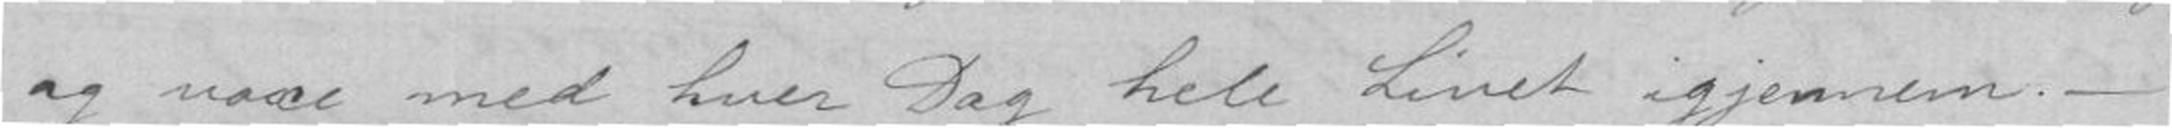og voxe med hver Dag hele Livet igjennem. -
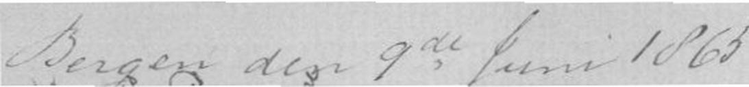Bergen den 9de Jumi 1865
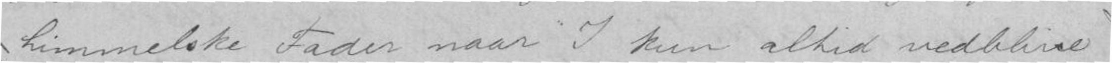himmelske Fader naar I kun altid vedblive
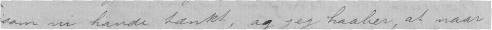som vi havde tænkt, og jeg husker, at naar
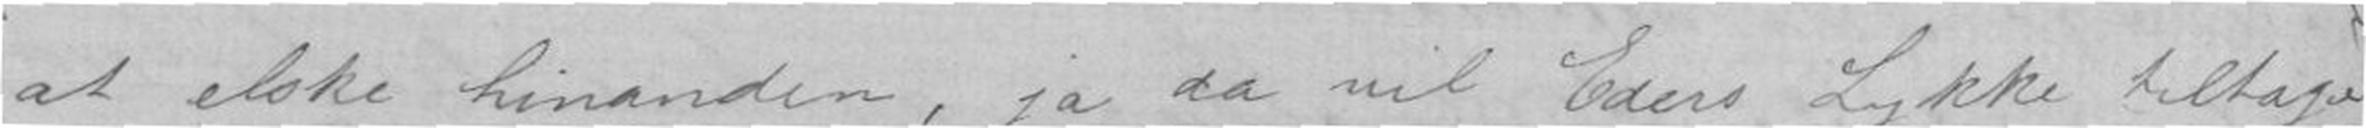at elske hinanden, ja da vil Eders Lykke tiltage
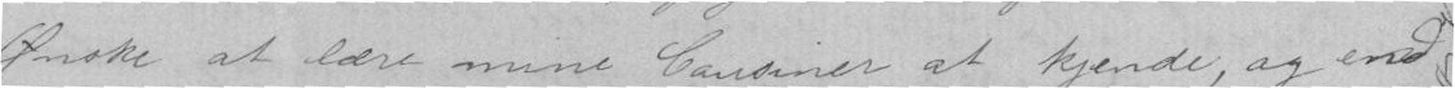Ønske at lære mine Cousiner at kjende, og end
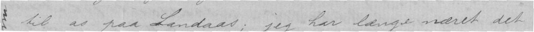til os paa Landaas; jeg har længe næret det
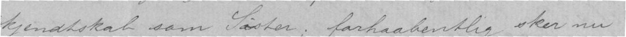kjendtskab som Søster; forhaabentlig sker nu
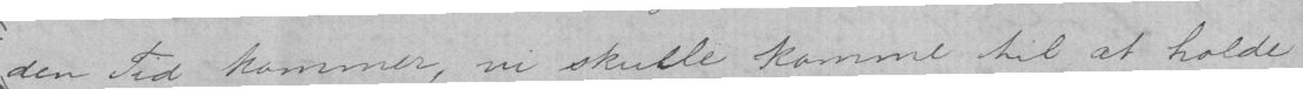den Tid kommer, vi skulle komme til at holde
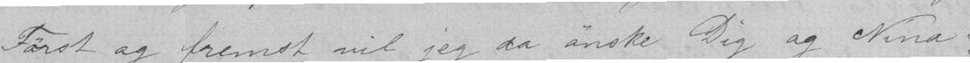Først og fremst vil jeg da ønske Dig og Nina
